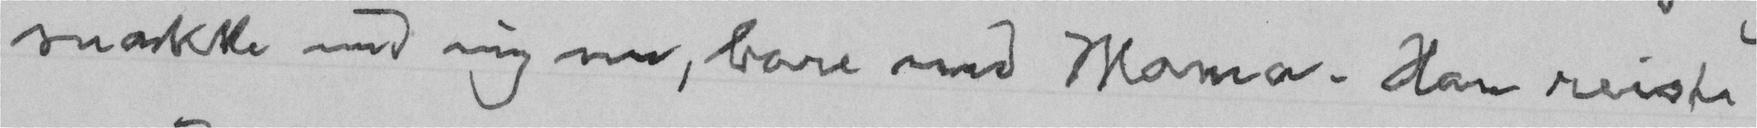snakke med mig nu, bare med Mama. Han reiste
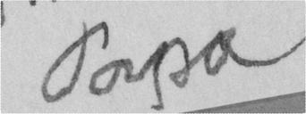Papa
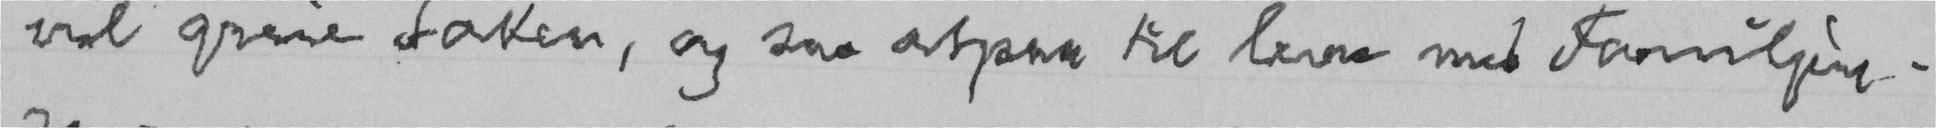vil greie Saken, og saa atpaa til leve med Familjen.
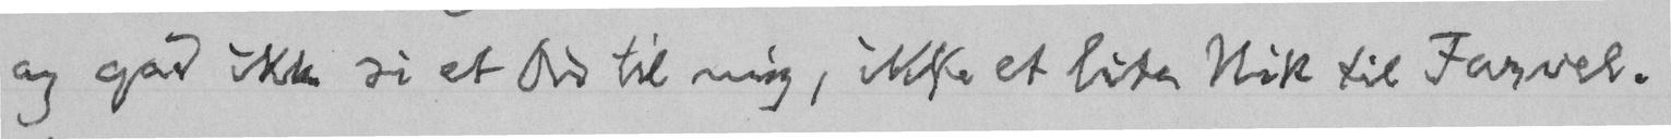og gad ikke si et Ord til mig, ikke et lite Nik til Farvel.
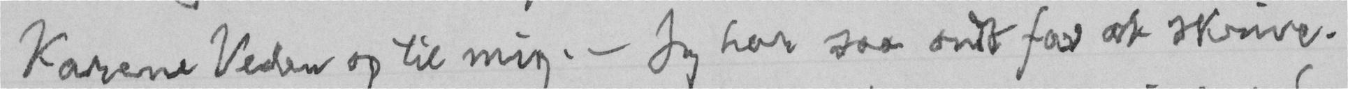Karene Veden op til mig. - Jeg har saa ondt for at skrive.
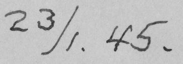23/1. 45.
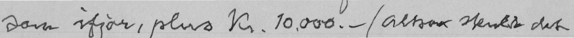som ifjor, plus Kr. 10.000. - (altsaa skulde det
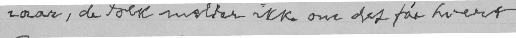iaar, de Folk mælder ikke om det før hvert
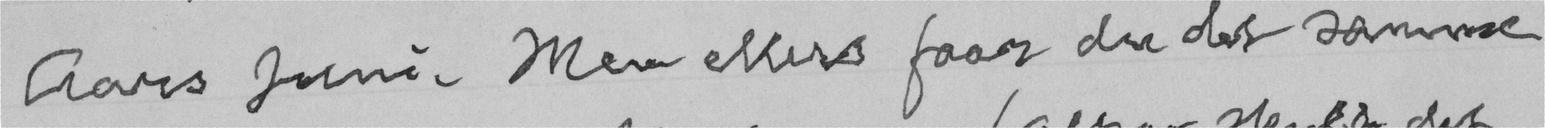Aars Juni. Men ellers faar du det samme
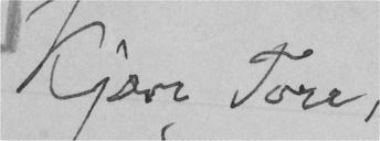Kjære Tore,
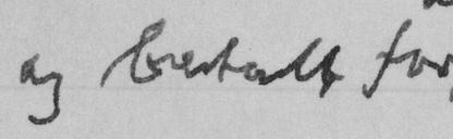og betalt for
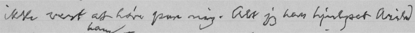ikke vert at høre paa mig. Alt jeg har hjulpet Arild
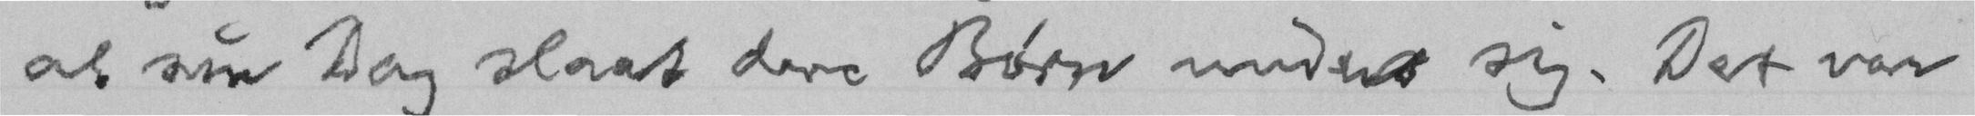al sin Dag slaat dere Børn under sig. Det var
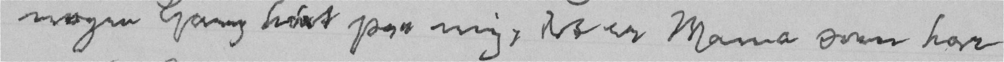nogen Gang hørt paa mig, det er Mama som har
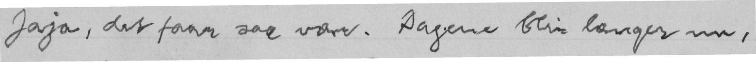Jaja, det faar saa være. Dagene blir længer nu,
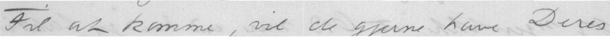til at komme, vil de gjerne have Deres
